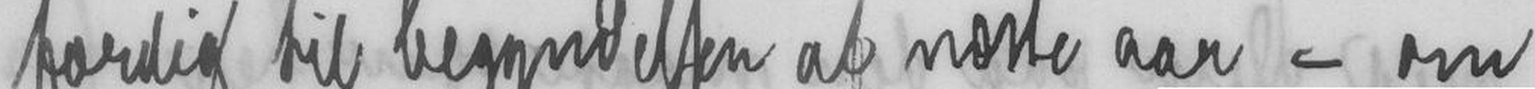færdig til begyndelsen af næste aar - om
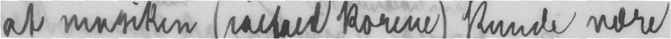at musiken (ialtfald korene) kunde være
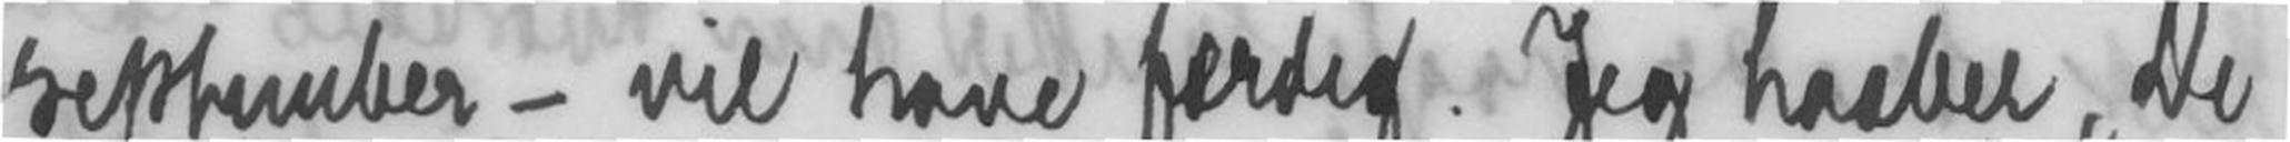september - vil have færdig. Jeg haaber De
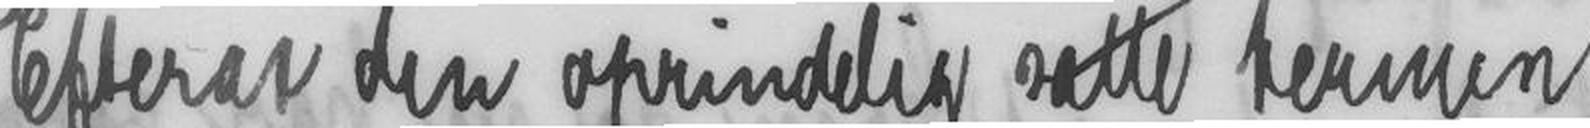Efterat den oprindelig satte termin
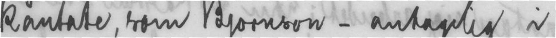kantate, som Bjørnson - antagelig i
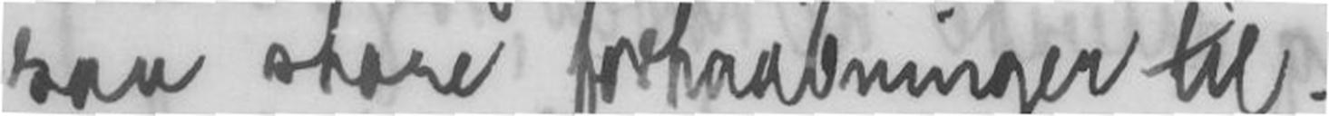saa store forhaabninger til.
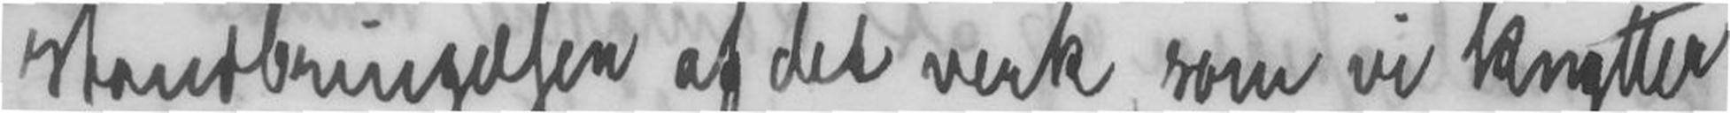standbringelsen af det verk, som vi knytter
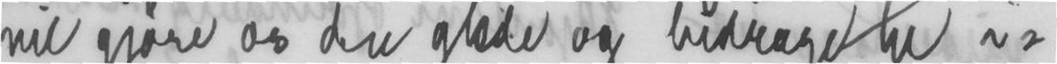vil gjøre os den glæde og bidrage til i-
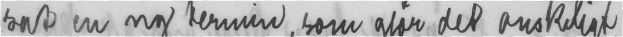sat en ny termin, som gjør det ønskeligt,
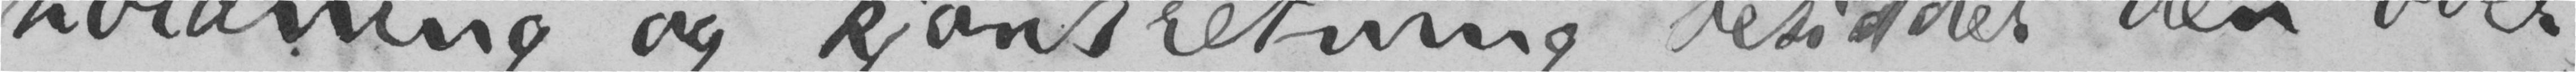holdning og kjønsretning besidder den over-
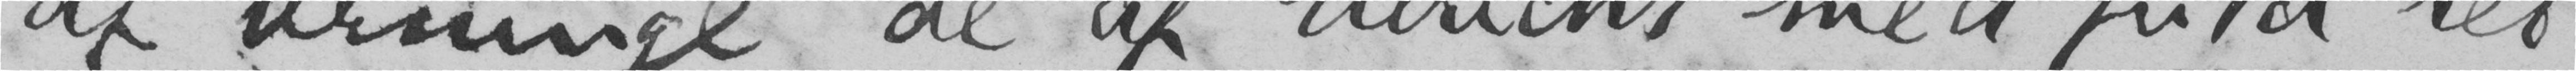af urninge, de af Ulrichs med fuld ret
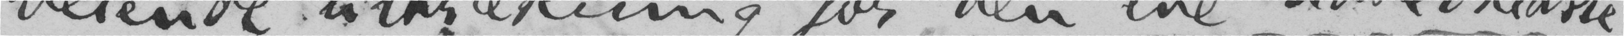veiende tiltrækning, for den ene hovedklasse
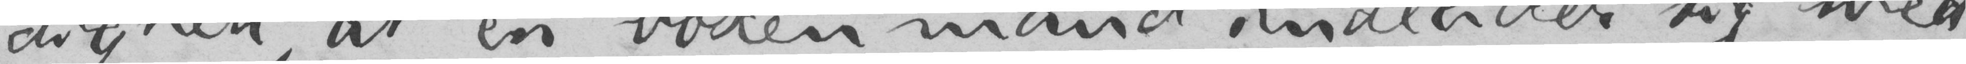dighed, at en voxen man indlader sig med
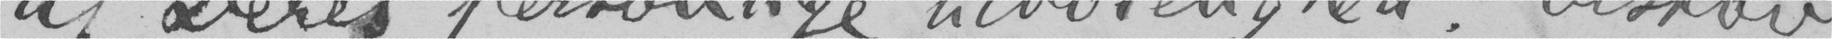af Deres personlige tilbøielighed. Elskov
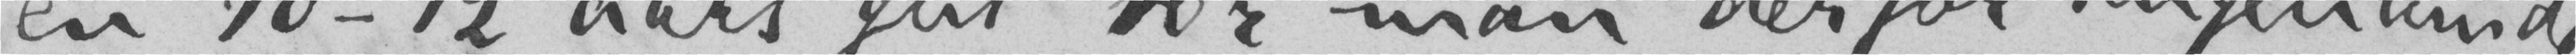en 10-12 aars gut tør man derfor ingelunde
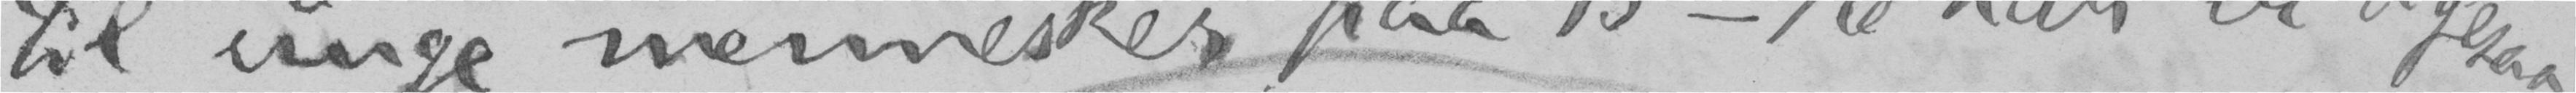til unge mennesker paa 15-16 aar er ligesaa
